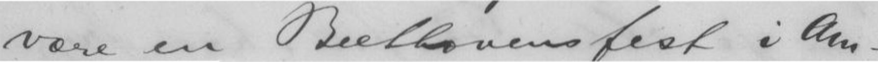være en Beethovensfest i Am-
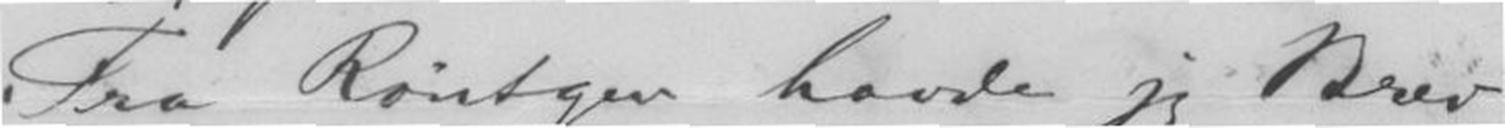Fra Röntgen havde jeg Brev
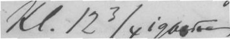Kl. 12 3/4 igaar.
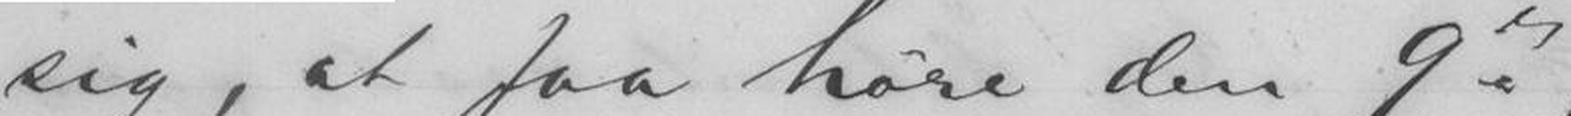sig, at faa høre den 9de,
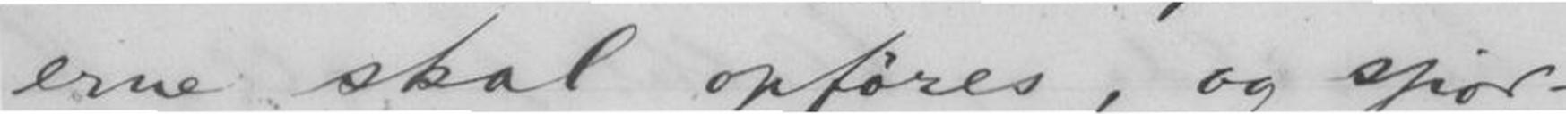erne skal opføres, og spør-
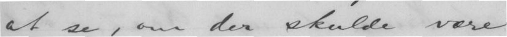at se, om der skulde være
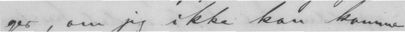ger, om jeg ikke kan komme
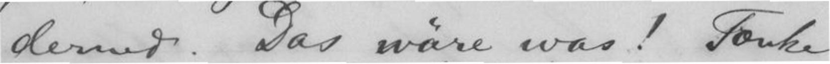derned. Das wäre was! Tænke
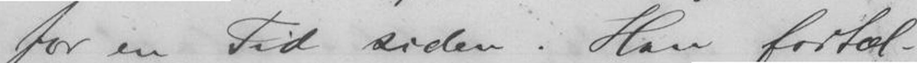for en Tid siden. Han fortæl-
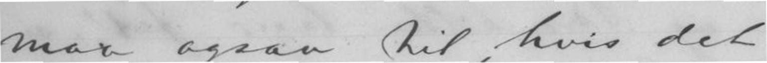maa ogsaa til, hvis det
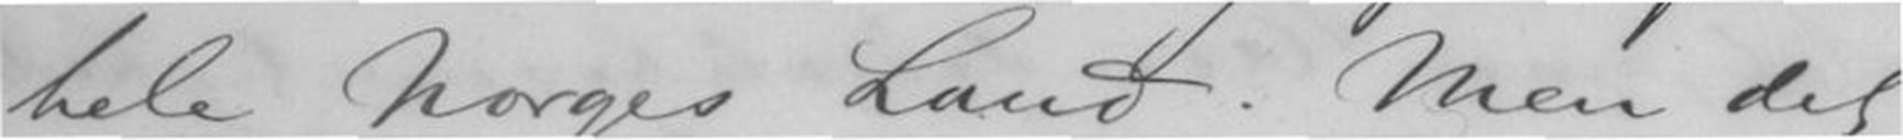hele Norges Land. Men det
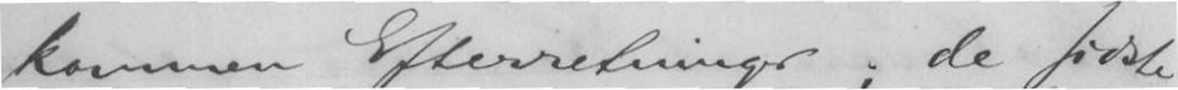kommen Efterretninger; de sidste
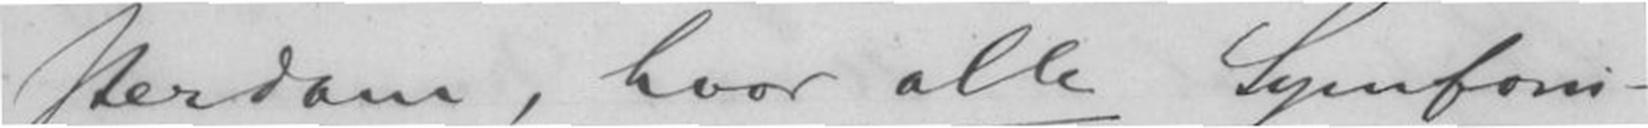sterdam, hvor alle Symfoni-
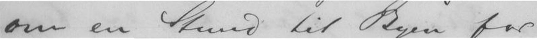om en Stund til Byen for
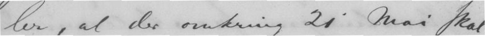ler, at der omkring 21 Mai skal
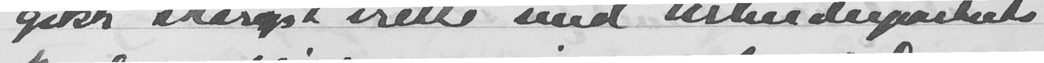gikk skarpt irette med Arbeiderpartiets
